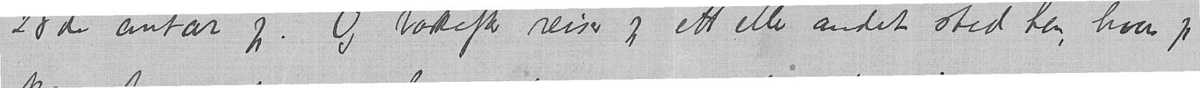28de antar jeg. Og bakefter reiser jeg ett eller andet sted hen, hvor jeg
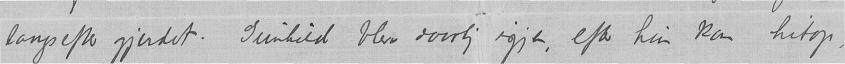langsefter gjerdet. Gunhild blev daarlig igjen, efter hun kom hitop,
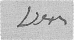Deres
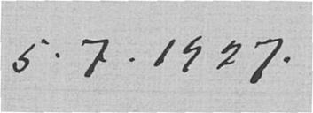5. 7. 1927.
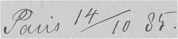Paris 14/10 85.
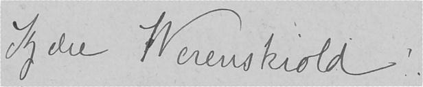Kjære Werenskiold!
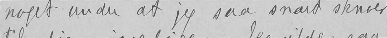noget under, at jeg saa snart skriver
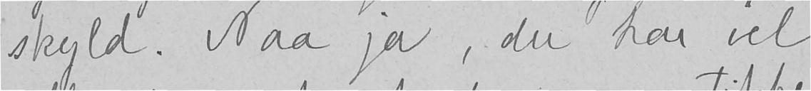skyld. Naa ja, du har vel
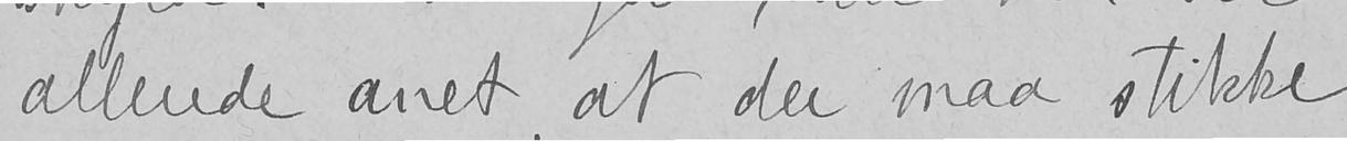allerede anet, at der maa stikke
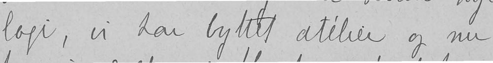logi, vi har byttet atelier og nu
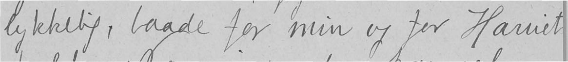lykkelig, baade for min og for Harriet
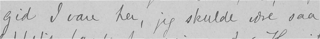gid I vare her, jeg skulde være saa
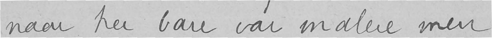naar her bare var malere, men
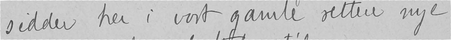sidder her i vort gamle rettere nye
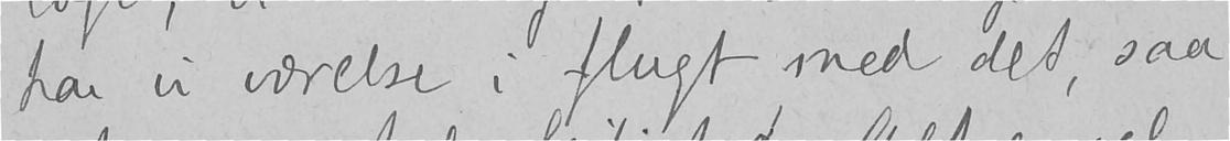har vi værelse i flugt med det, saa
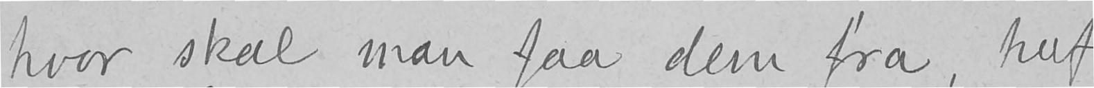hvor skal man faa dem fra, huf
